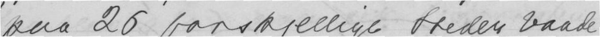paa 26 forskjellige steder baade
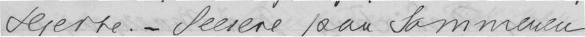Hjerte.- Senere paa Sommeren
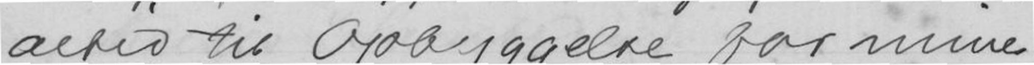altid til Opbyggelse paa mine
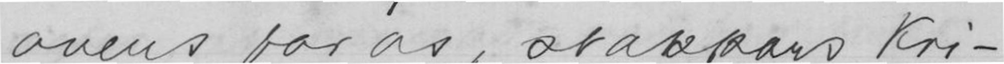overs for os, stakkars Kri-
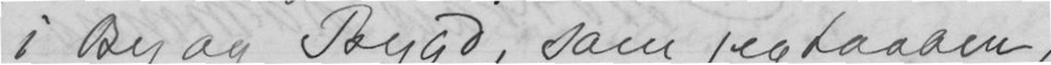i By og Bygd, som jeg haaber,
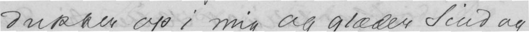dukker op i mig og glæder Sind og
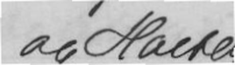og Holder
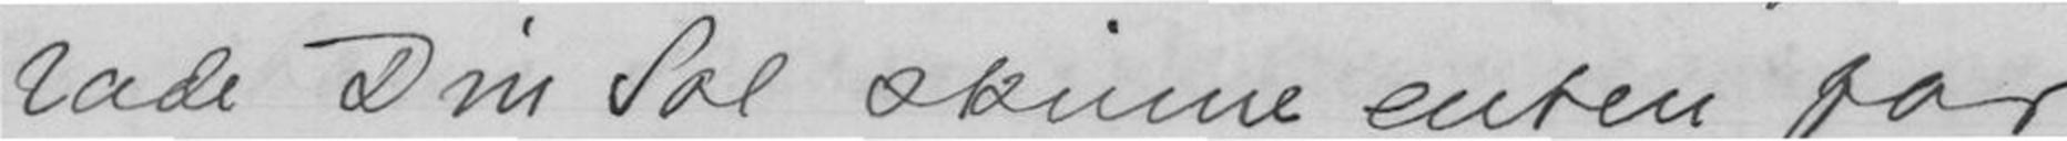lade Din Sol skinne enten for
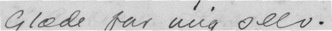Glæde for mig selv.
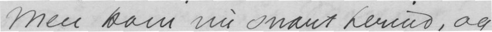Men kan nu snart herind, og
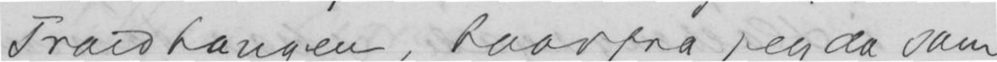Troldhaugen, hvorfra jeg da som
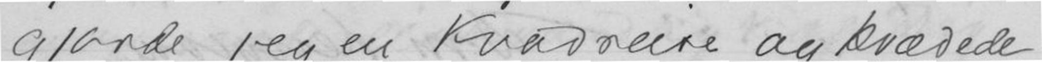gjorde jeg en Kvadserie og kvædede
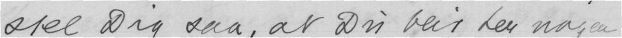Hel Dig saa, at Du blir her nogen
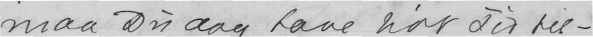maa Du dog hav hør Tid til-
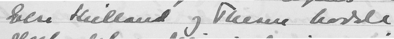Else Kielland og Therese hadde
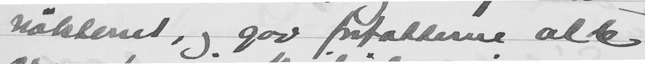nøkternt, og gav fortatterne all
